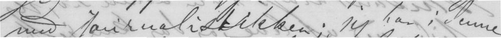med journalistikken; jeg har i denne
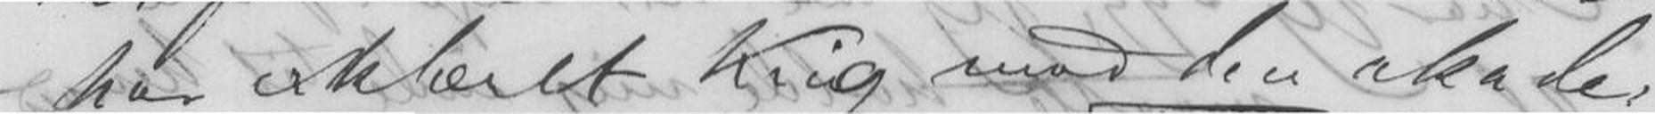har erklæret Krig mod den akade-
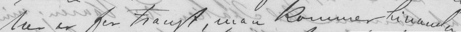her er for trangt, man kommer hinnanden
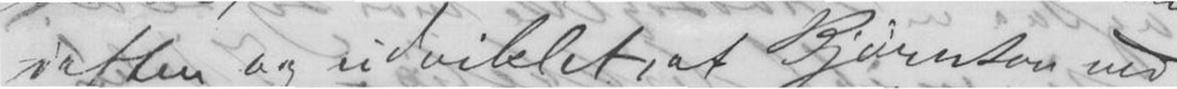iaften og udviklet, at Bjørnson ved
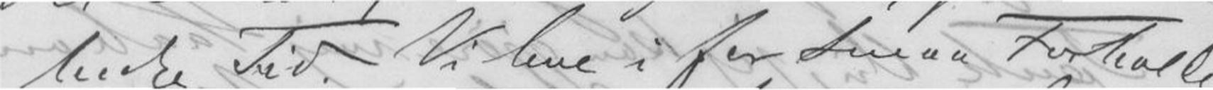bedre Tid. Vi leve i for smaa Forholde,
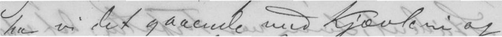har vi det gaaende med Kjævleri og
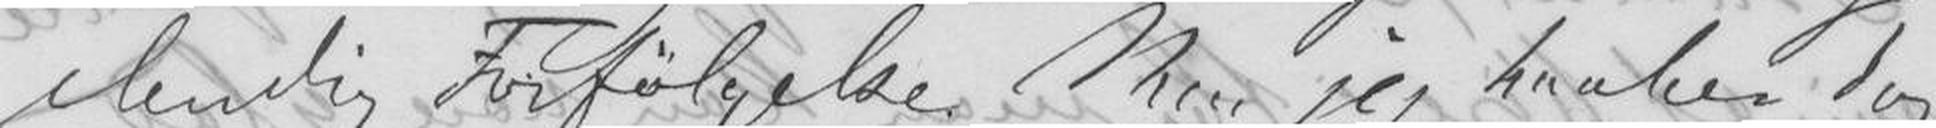elendig Forfølgelse. Men jeg haaber dog,
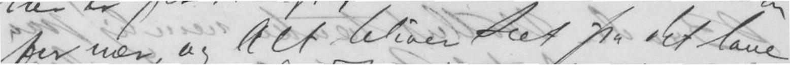for nær, og Alt bliver sæt fra det lave
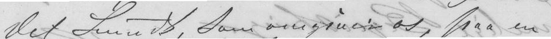det Smuds, som omgiver os, paa en
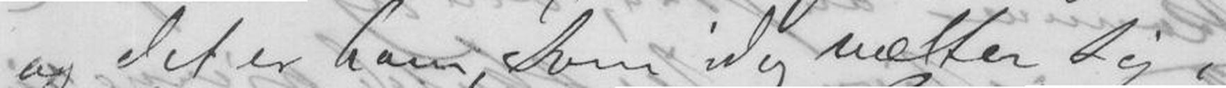og det er ham, som idag mætter sig
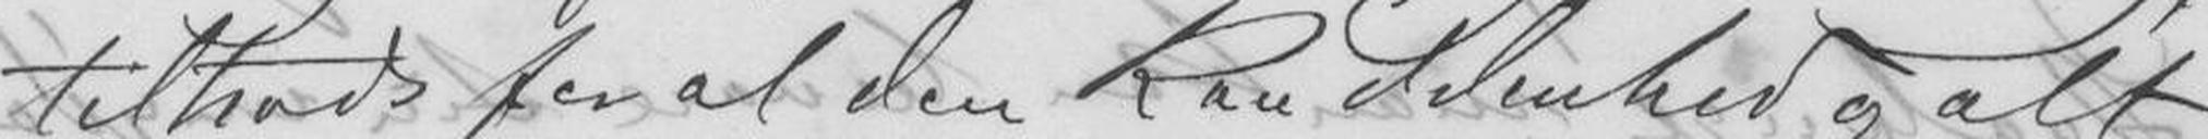tiltrods for al den Raaddenhed og alt
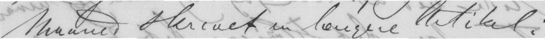Maaned skrevet en længere Artikel i
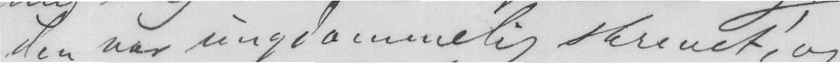den var ungdommelig skrevet, og
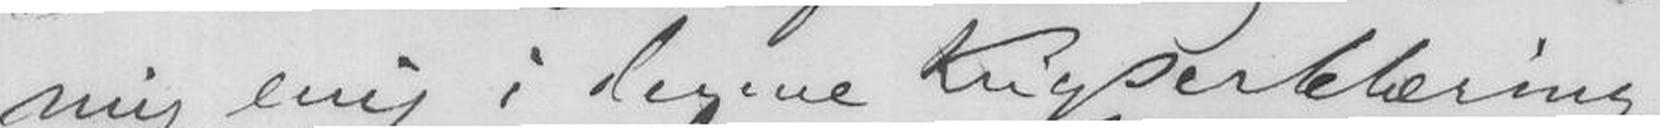mig enig i denne Krigserklæring;
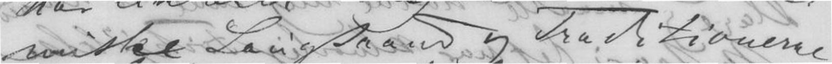minske Laugsaand og Traditionerne
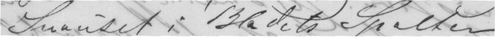Snauset i Bladets Spalter.
